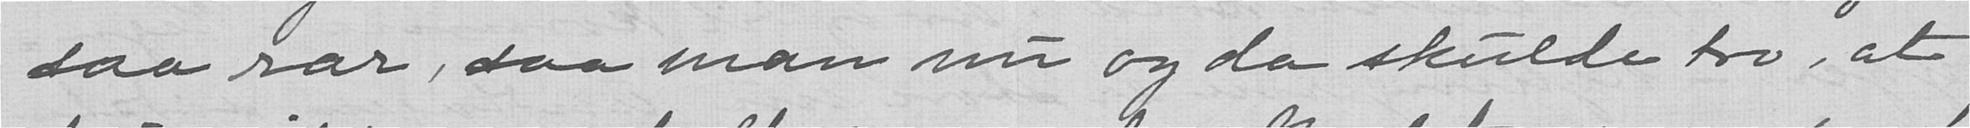saa rar, saa man nu og da skulde tro, at
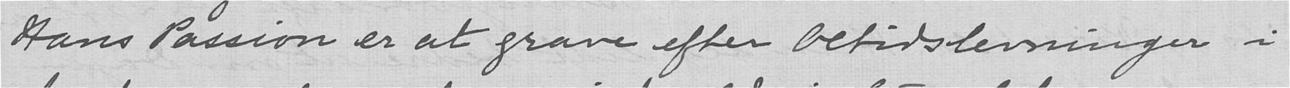Hans Passion er at grave efter Oltidslevninger i
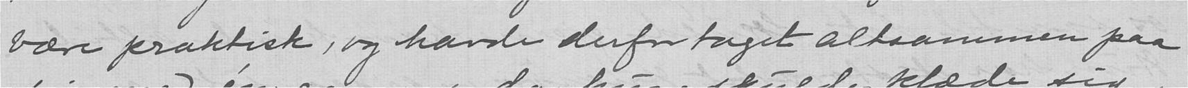være praktisk, og havde derfor taget altsammen paa
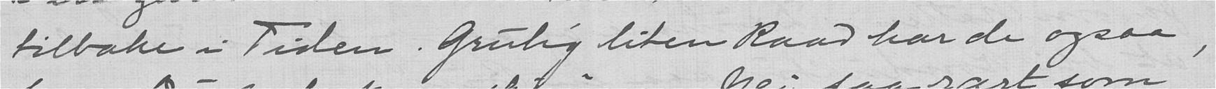tilbake i Tiden. Grulig liten Raad har de ogsaa,
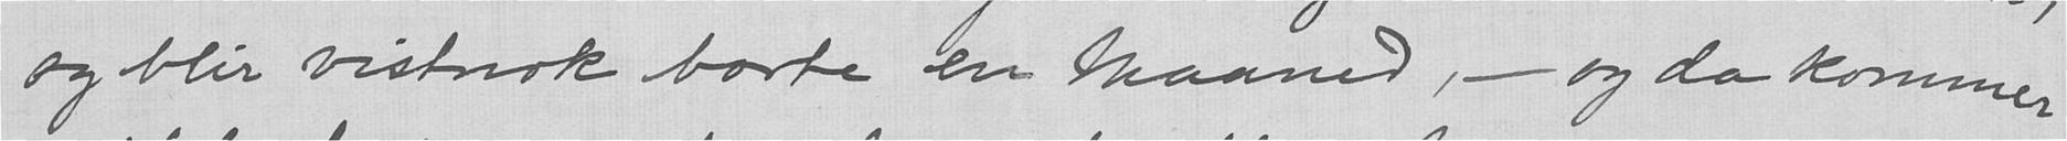og blir vistnok borte en Maaned, - og da kommer
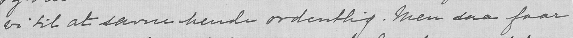vi til at savne hende ordentlig. Men saa faar
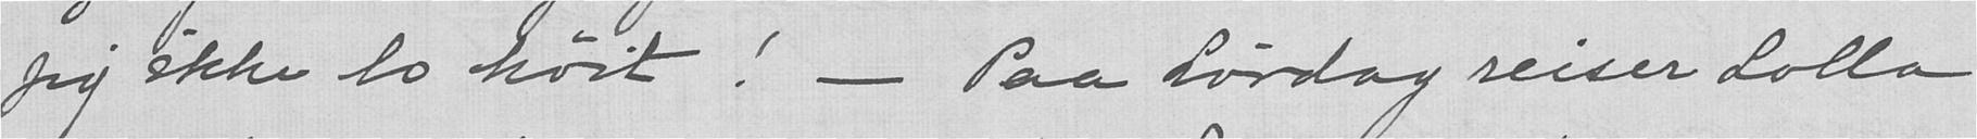jeg ikke lo høit! - Paa lørdag reiser Lolla
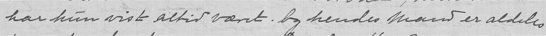har hun vist altid været. Og hendes Mand er aldeles
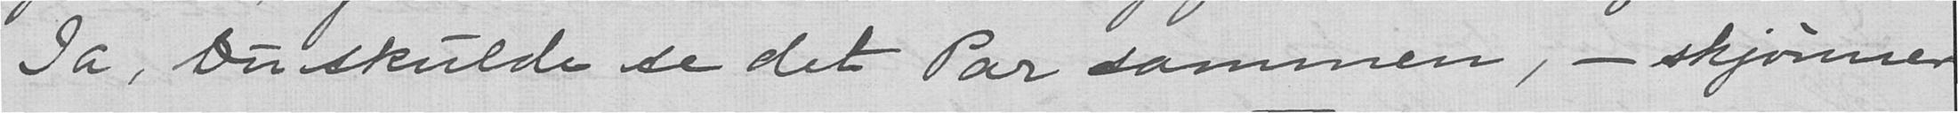Ja, Du skulde se det Par sammen, - skjønner
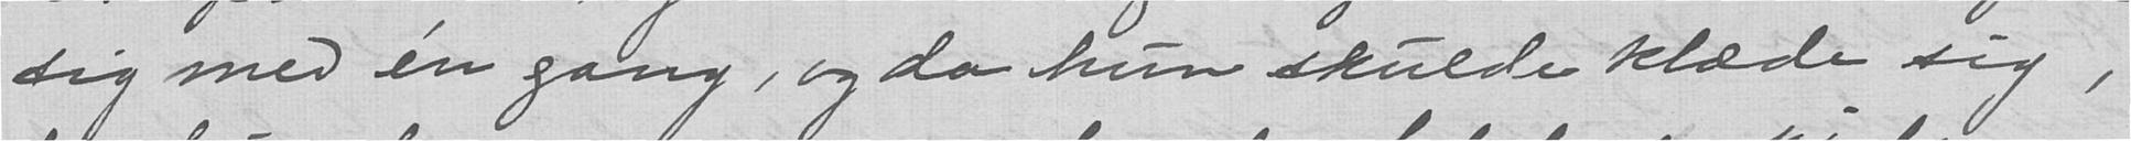sig med én gang, og da hun skulde klæde sig,
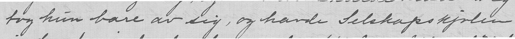tog hun bare av sig, og havde Selskapskjolen
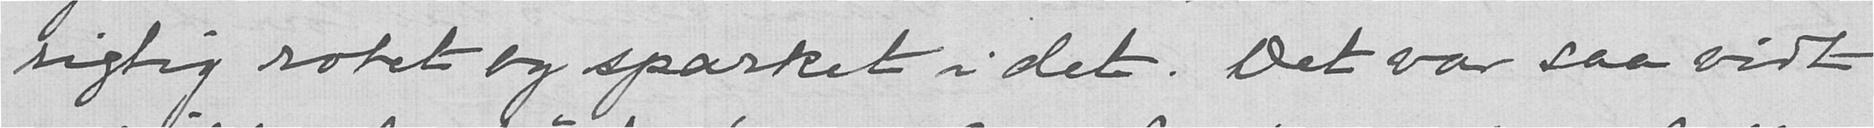rigtig rotet og sparket i det. Det var saa vidt
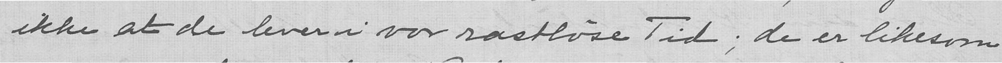ikke at de lever i vor rastløse Tid; de er likesom
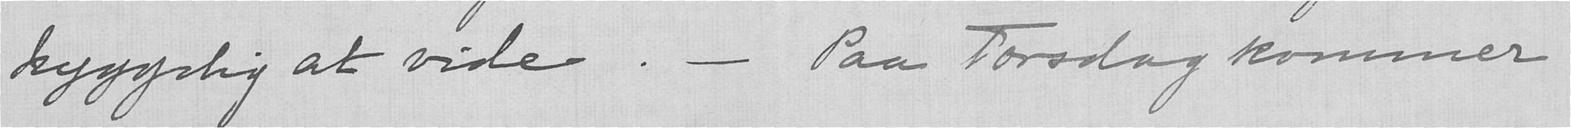hyggelig at vide. - Paa Torsdag kommer
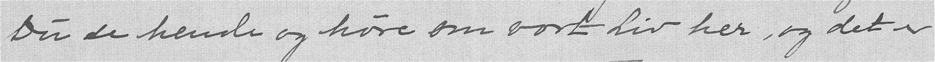Du se hende og høre om vort Liv her, og det er
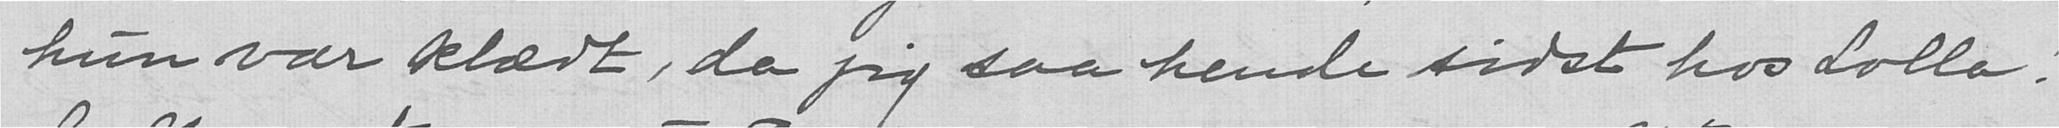hun var klædt, da jeg saa hende sidst hos Lolla!
